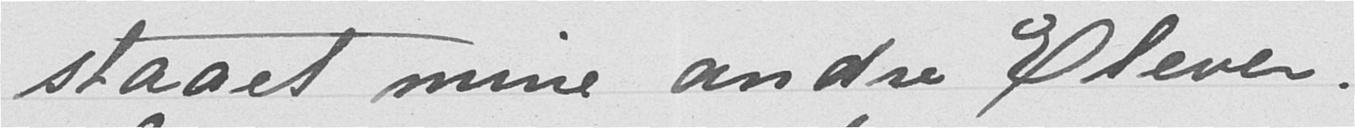staaet mine andre Elever.
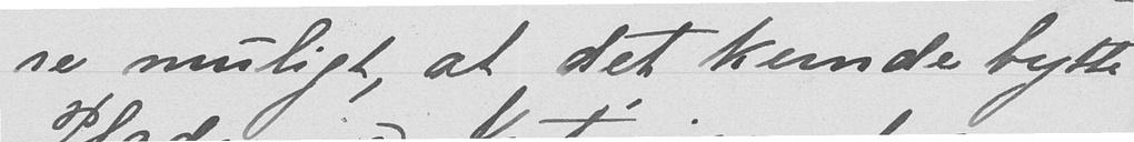re muligt, at det kunde bytte
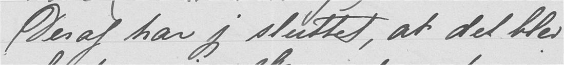Deraf har jeg sluttet, at det blev
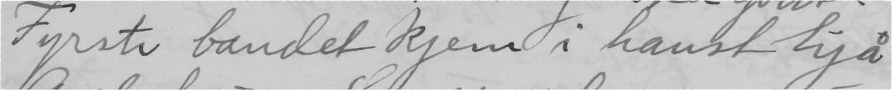Fyrste bandet kjem i haust hjå
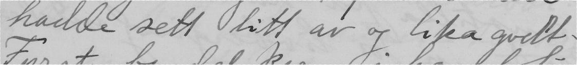hadde sett litt av og lika godt.
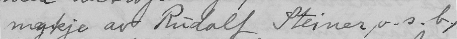mykje av Rudolf Steiner o.s.b.,
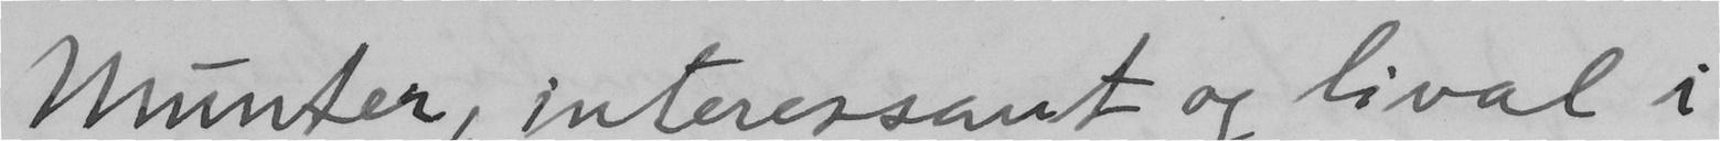munter, interessant og livat i
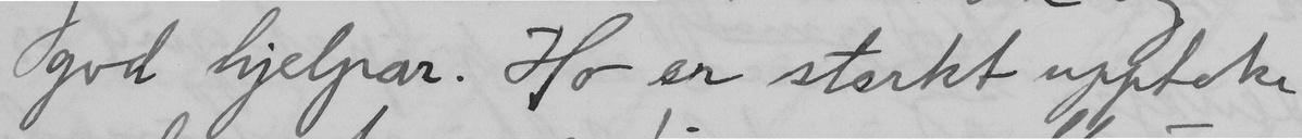god hjelpar. Ho er sterkt uppteke
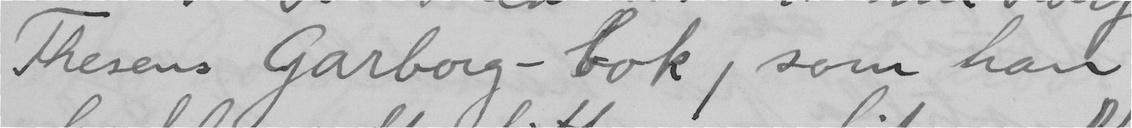Thesens Garborg-bok, som han
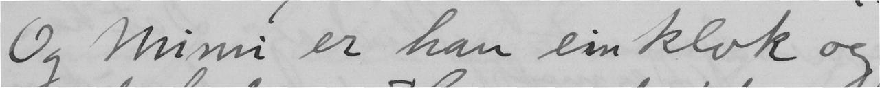Og mimi er han ein klok og
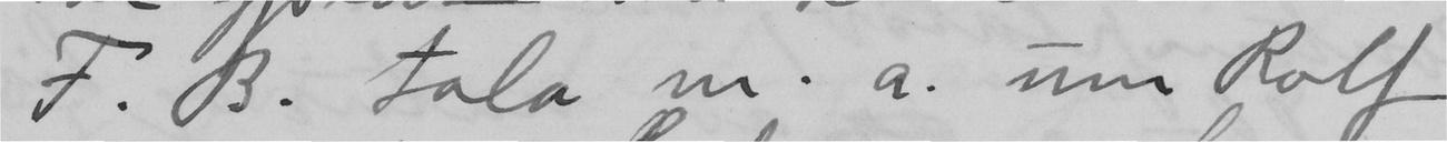F.B. tola m.a. um Rolf
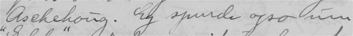Aschehoug. Eg spurde ogso um
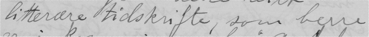litterære tidskrifte, som berre
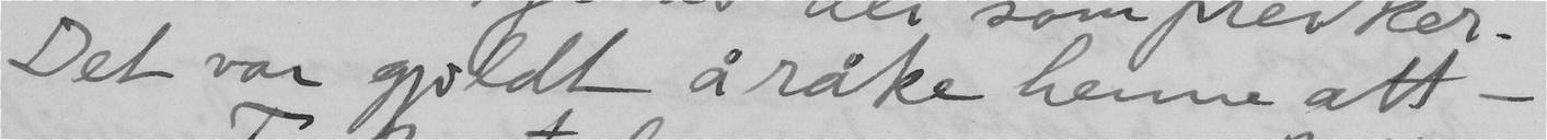Det var gjildt å råke henne att –
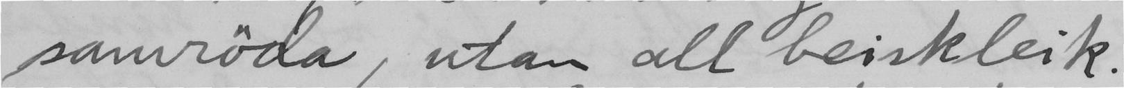samröda, utan all beiskleik.
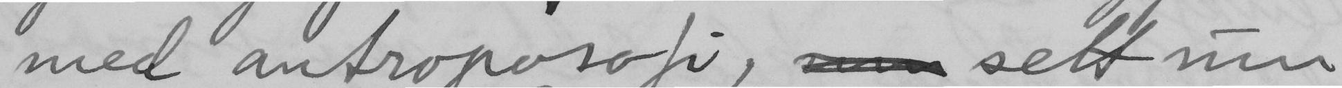med antroposofi,  sett um
